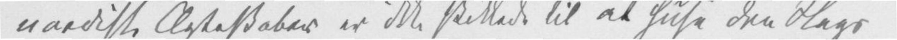nordiske Ægteskaber er ikke skikkede til at huse den Slags
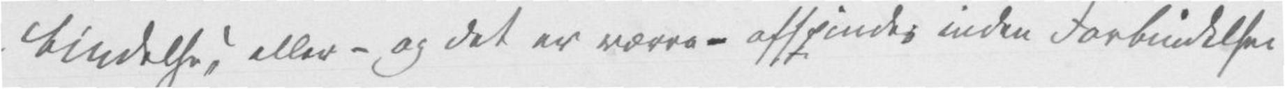bindelse, eller – og det er værre – affindes inden Forbindelsen
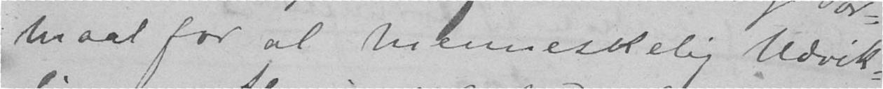maal for al menneskelig Udvik-
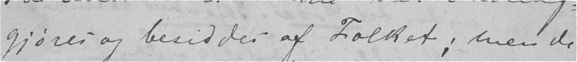gjøres og besiddes af Folket; men de
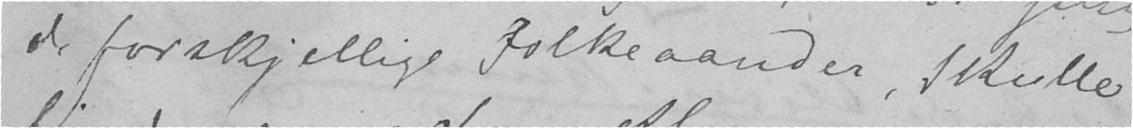de forskjellige Folkeaander, skulle
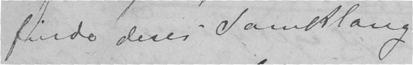finde deres Samklang
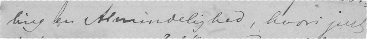ling en Almindelighed, hvori just
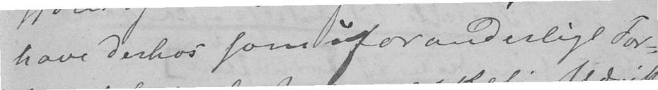have derhos som uforanderlige For-
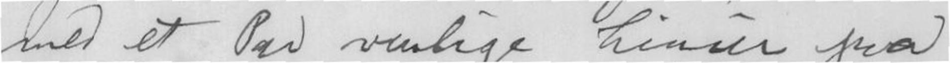med et Par venlige Linier paa
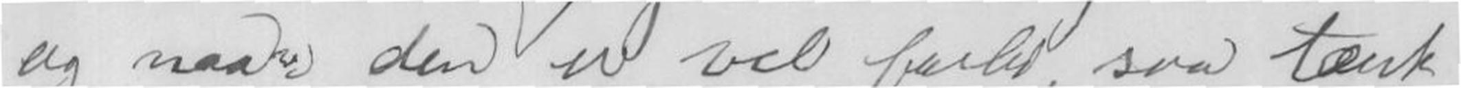og naar den er vel forbi, saa tænk
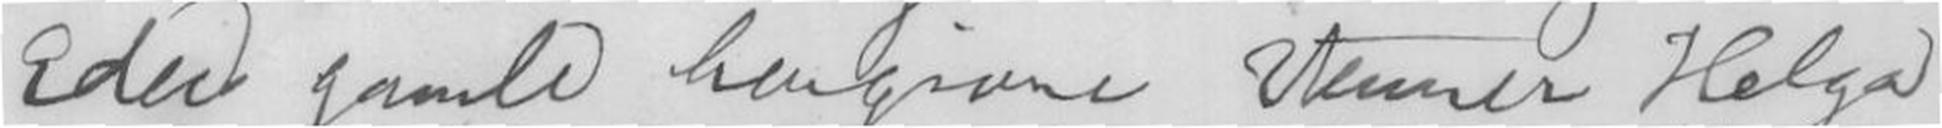Eders gamle hengivne Venner Helga
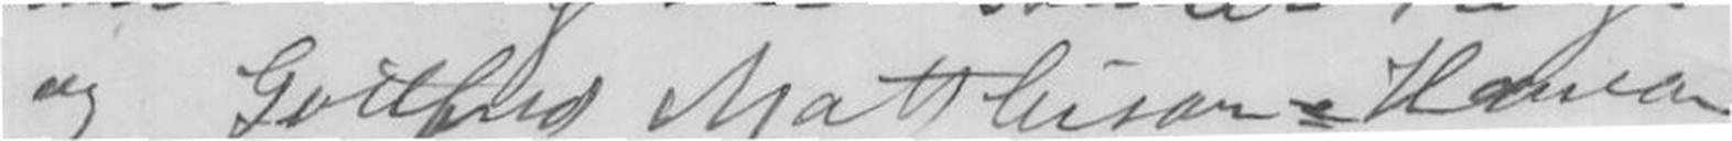og Gottfred Matthison=Hansen
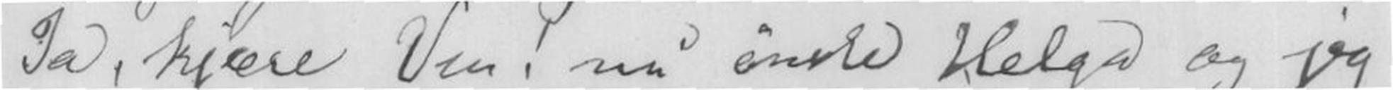Ja, kjære Ven ! nu ønske Helga og jeg
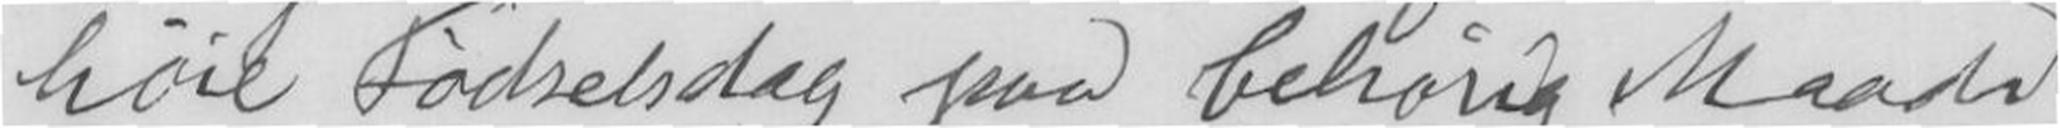høie Fødselsdag paa behørig Maade
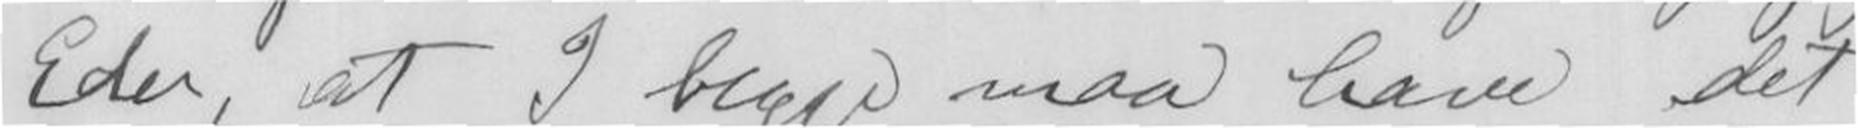Eder, at I begge maa have det
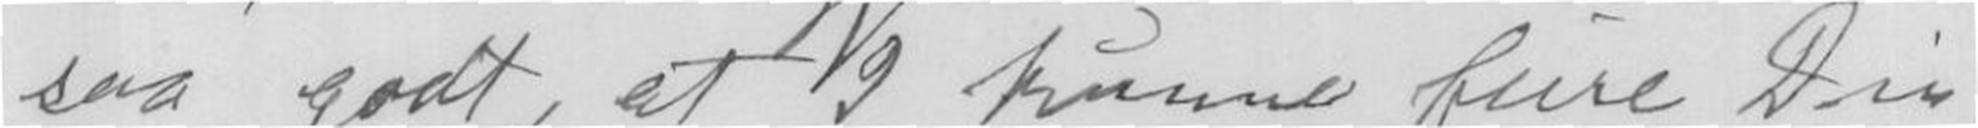saa godt, at I kunne feire Din
